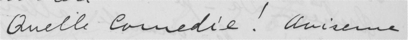Quelle Comedie. Aviserne
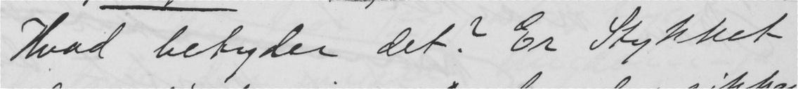Hvad betyder det? Er Stykket
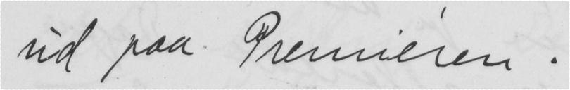ud paa Premieren.
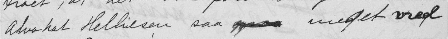Advokat Helliesen saa  meget vred
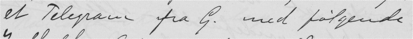et Telegram fra G. med følgende
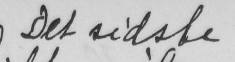Det sidste
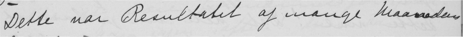Dette var Resultatet af mange Maaneders
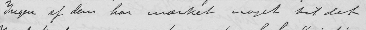Ingen af dem har mærket noget til det
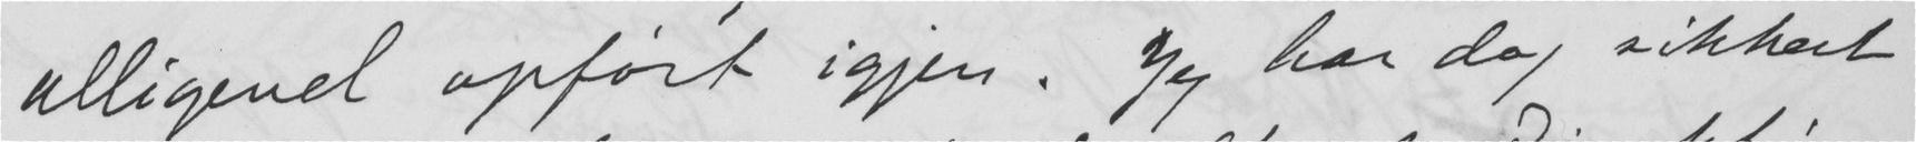alligevel opført igjen. Jeg har dog sikkert
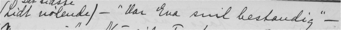(Lidt nølende) — "Var Eva snil bestandig" -
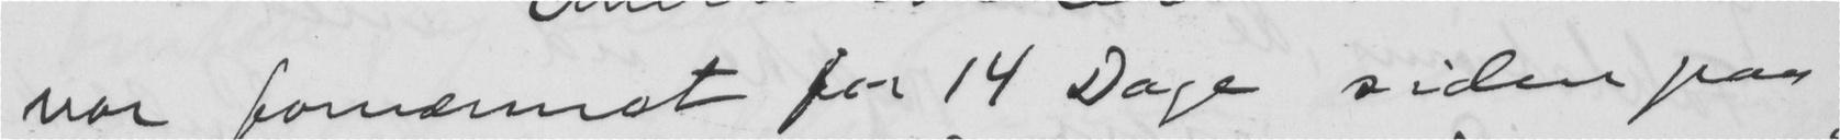var fornærmet for 14 Dage siden paa
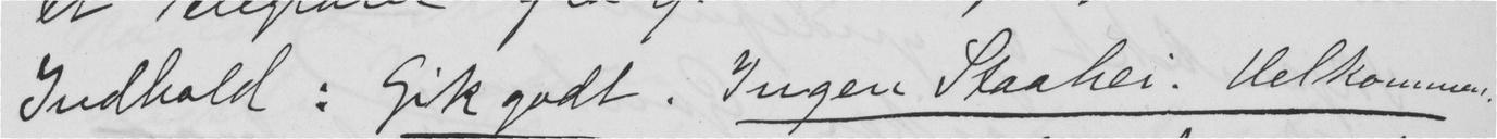Indhold: Gik godt. Ingen Staahei. Velkommen.
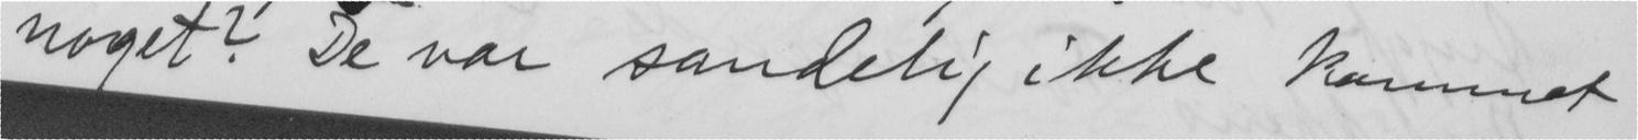noget? De var sandelig ikke kommet
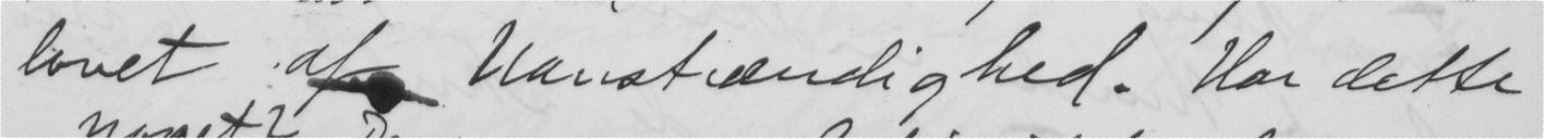lovet af Uanstændighed. Har dette
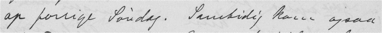op forrige Søndag. Samtidig kom ogsaa
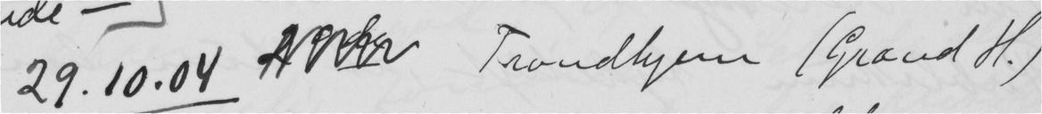29.10.04  Trondhjem (Grand H.)
